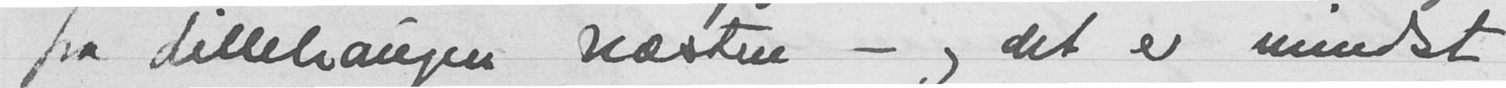fra Lillehaugen næsten - og det er mindst
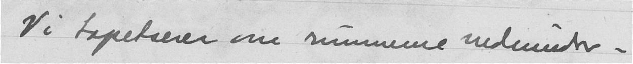Vi tapetserer om rummene nedenunder -
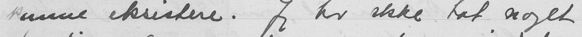kunne eksistere. Jeg har ikke tat noget
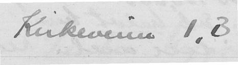Kirkeveien 1, B
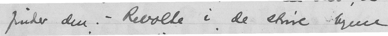finder den. - Revolte i de større byene
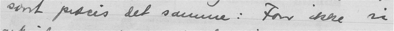svart præcis det samme: Faar ikke vi
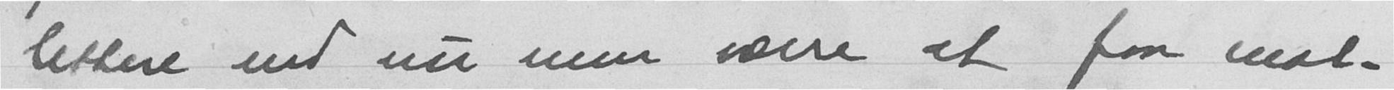lettere end nu men værre at faa mat -
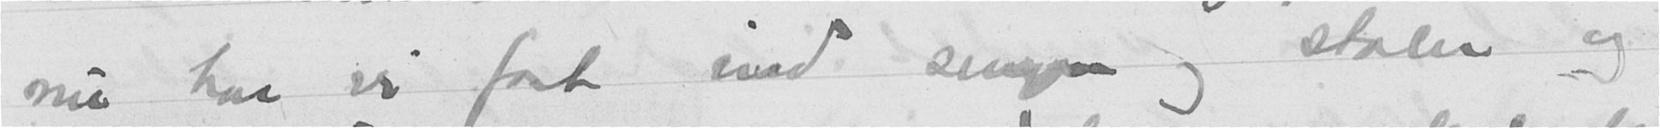nu har vi faat ind sengen og stolen og
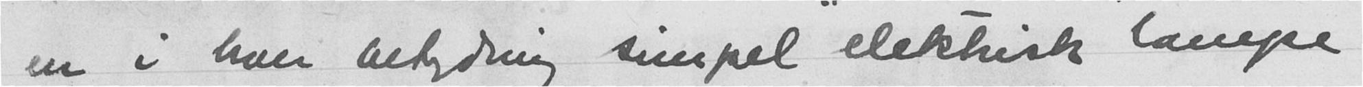en i hver betydning simpel elektrisk lampe
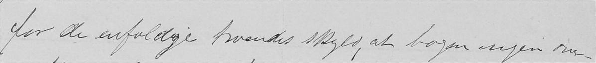for de enfoldige troendes skyld, at bogen ingen over-
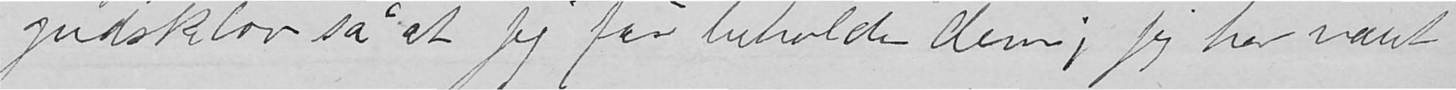gudskelov så at jeg får beholde dem; jeg har været
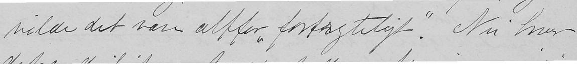vilde det være altfor "fortryteligt". Nei hvor
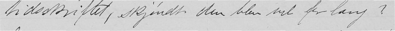tidsskriftet, skjøndt den blev vel for lang?
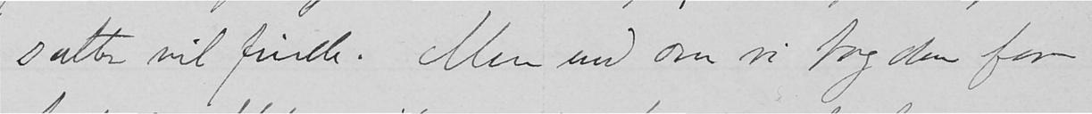sætter vil finde. Men end om vi tog den for
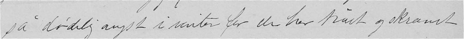så dødelig angst i vinter for de har hørt og skræmt
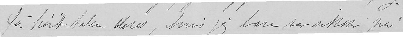få hørt talen deres, hvis jeg bare var sikker på
